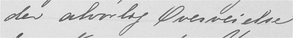der alvorlig Overveielse
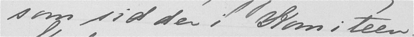som sidder i Komiteen.
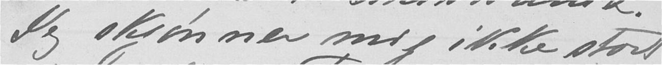Jeg skjønner mig ikke stort
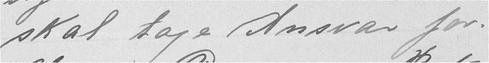skal tage Ansvar for.
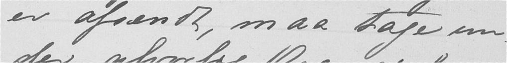er afsendt, maa tage un-
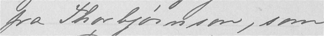fra Thorbjørnson, som
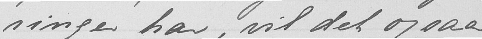ringer har, vil det ogsaa
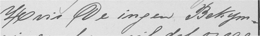Hvis De ingen Bekym-
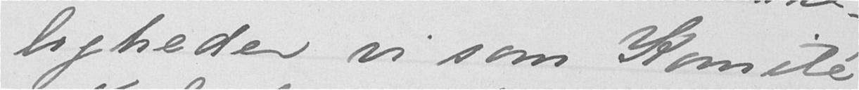ligheder vi som Komité
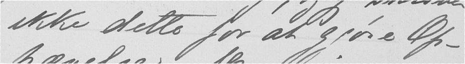ikke dette for at gjøre Op-
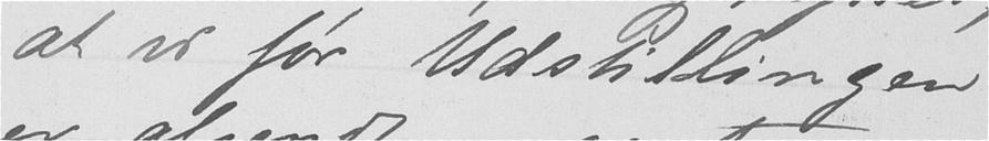at vi før Udstillingen
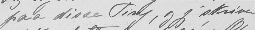paa disse Ting, og jeg skriver
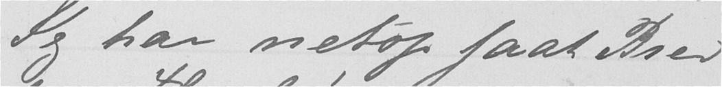Jeg har netop faat Brev
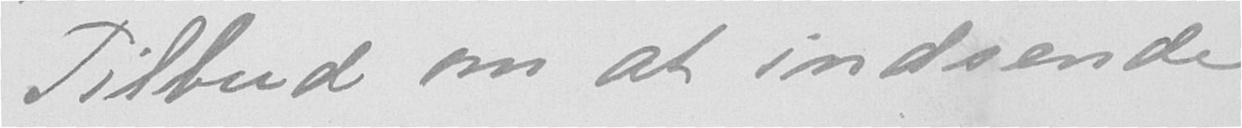Tilbud om at indsende
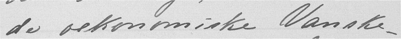de oekonomiske Vanske-
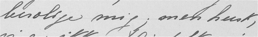berolige mig; men husk,
